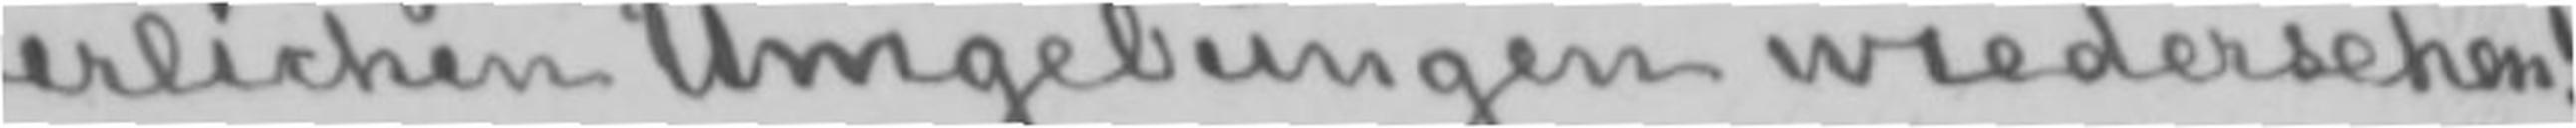terlichen Umgebungen wiedersehen!
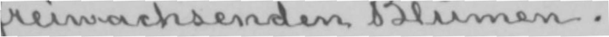freiwachsenden Blumen.
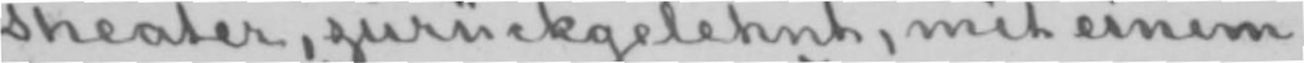Theater, zurückgelehnt, mit einem
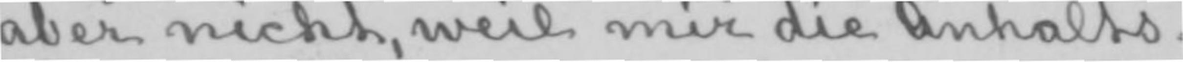aber nicht, weil mir die Anhalts-
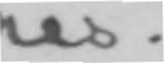res.
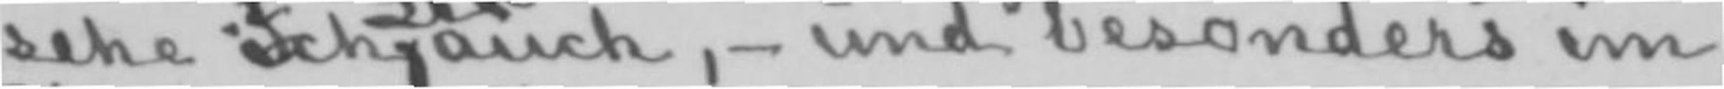sehe ich Sie auch,- und besonders im
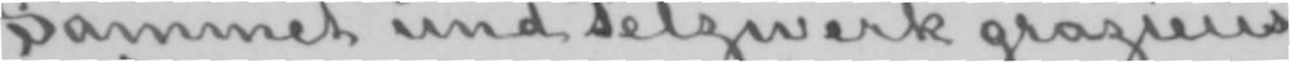Sammet und Pelzwerk grazieus
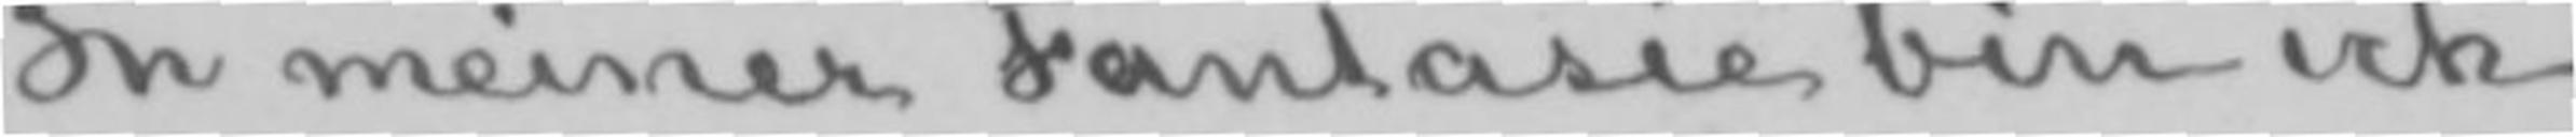In meiner Fantasie bin ich
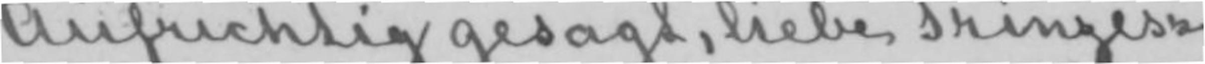Aufrichtig gesagt, liebe Prinzess-
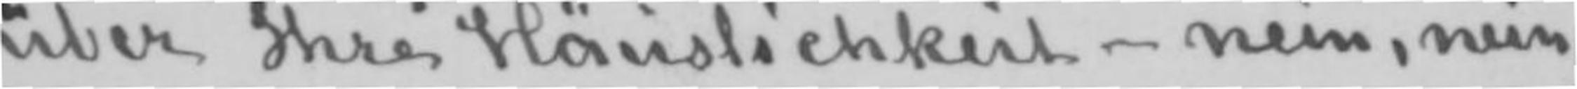über Ihre Häuslichkeit- nein, nein,
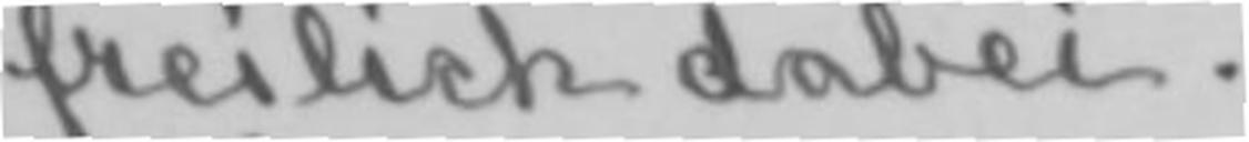freilich dabei.
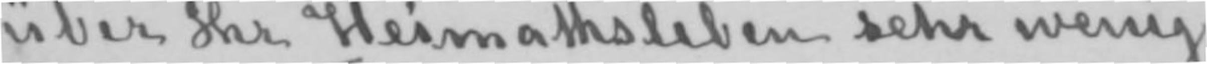über Ihr Heimathsleben sehr wenig
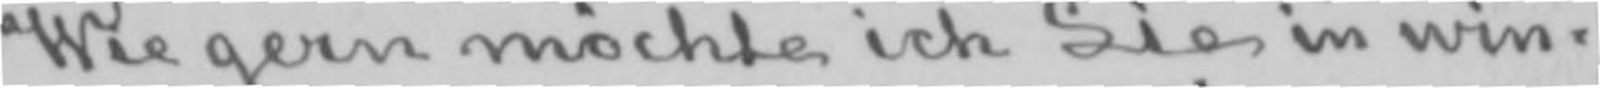Wie gern möchte ich Sie in win-
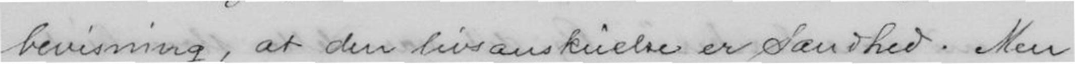bevisning, at den livsanskuelse er sandhed. Men
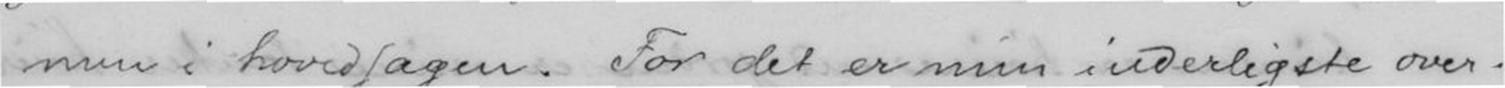men i hovedsagen. For det er min inderligste over-
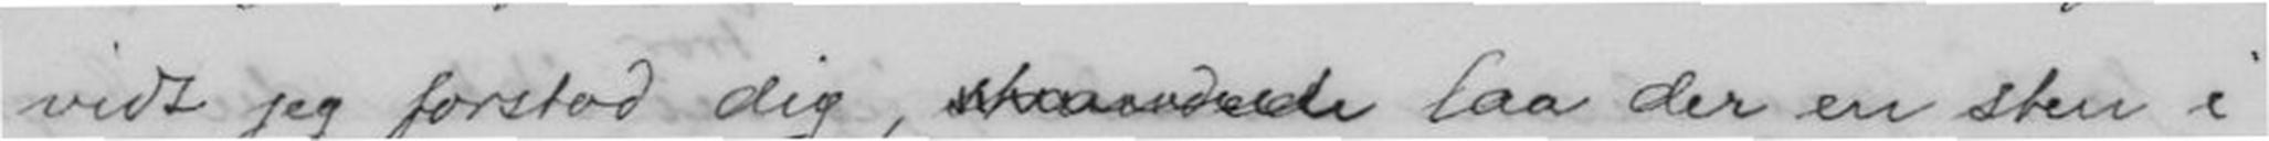vidt jeg forstod dig, laa der en sten i
